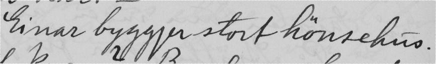Einar byggjer stort hönsehus.
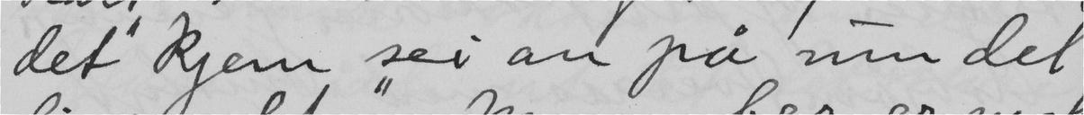det "kjem sei an på um det
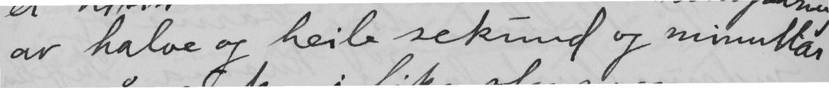av halve og heile sekund og minuttar
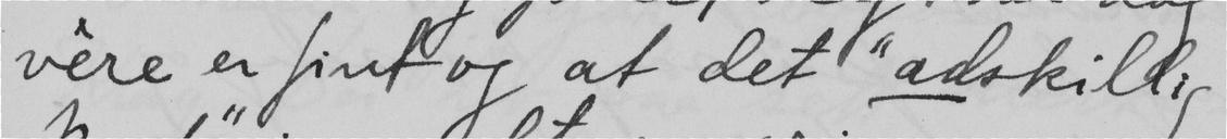vêre er fint og at det "adskillig
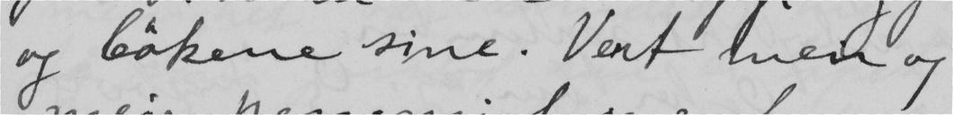og bökene sine. Vert meir og
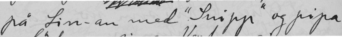på Lin-an med "Snipp" og pipa
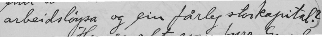arbeidslöysa og ein fårleg storkapital?
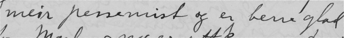meir pessemist og er berre glad
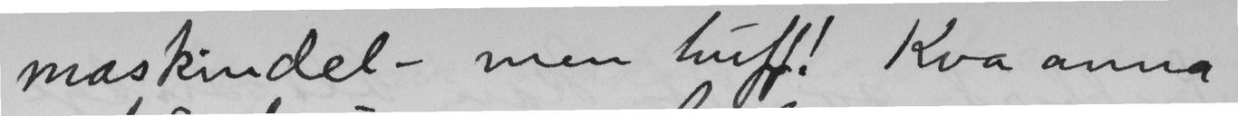maskindel – men huff! Kva anna
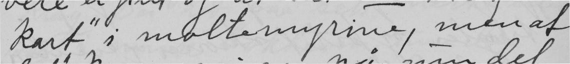kart" i moltemyrine, men at
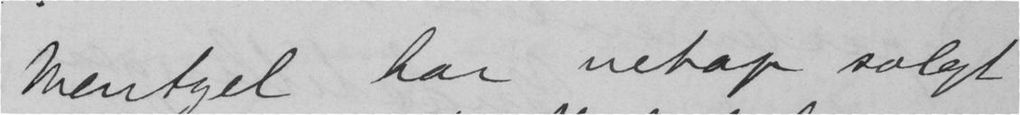Wentzel har netop solgt
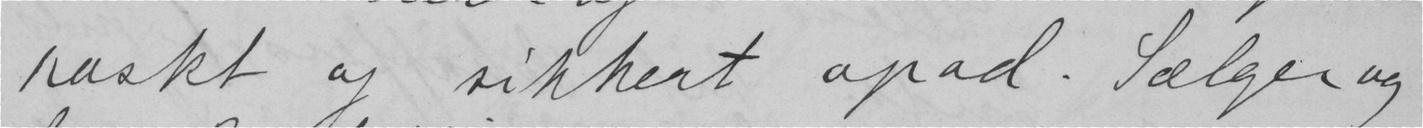raskt og sikkert opad. Sælger og
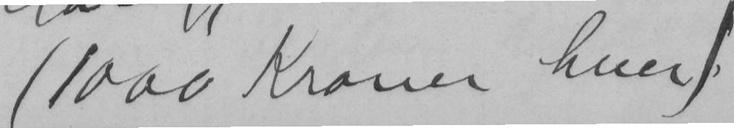(1000 Kroner hver)
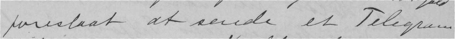foreslaat at sende et Telegram
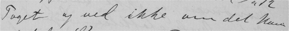Toget og ved ikke om det kom
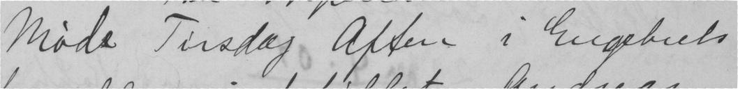møde Tirsdag Atten i Engebrets
